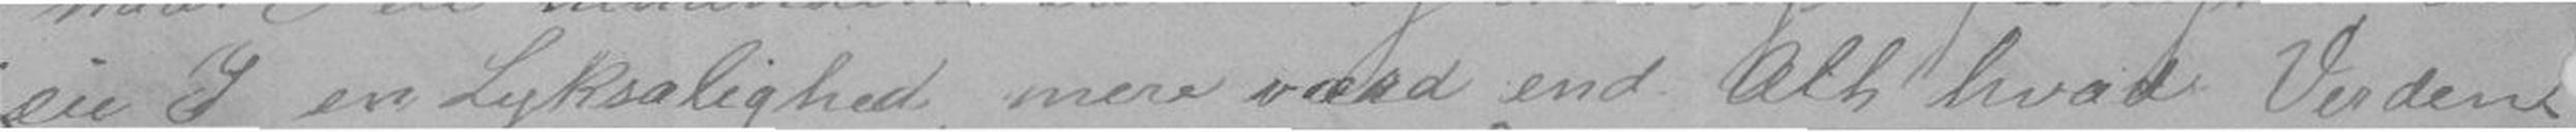eie I en Lyksalighed, mere værd end Alt hvad Verden
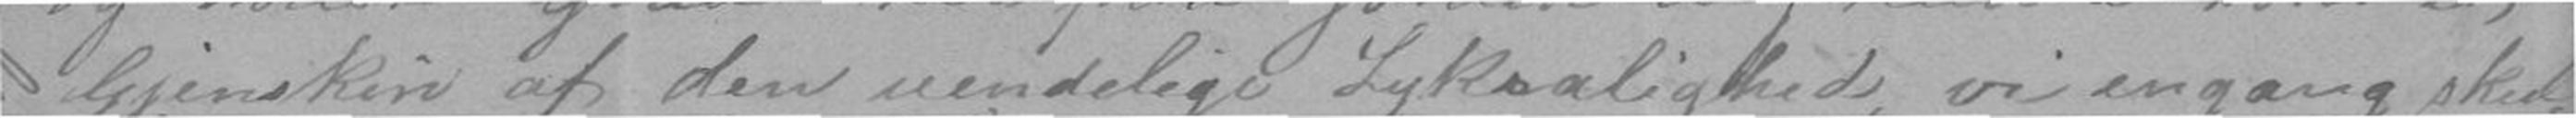Gjenskin af den uendelige Lyksalighed, vi engang skul-
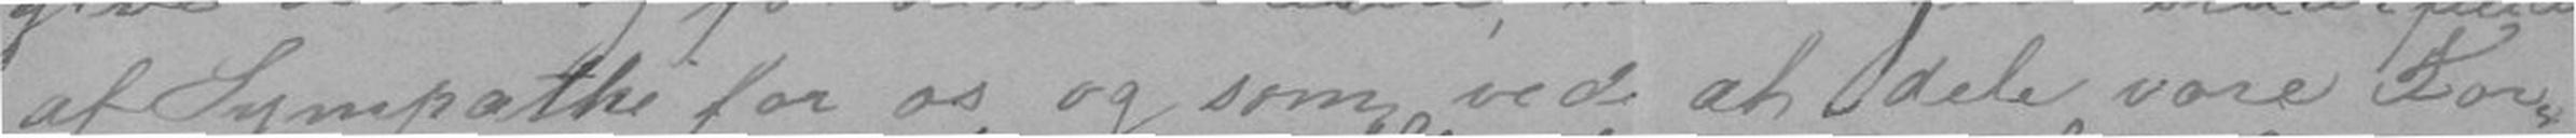af Sympathi for os, og som ved at dele vore For-
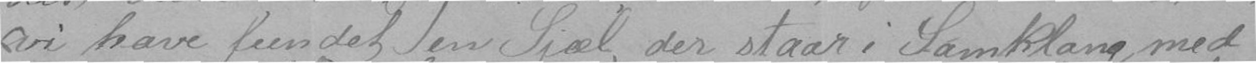vi have fundet en Sjæl, der staar i Samklang med
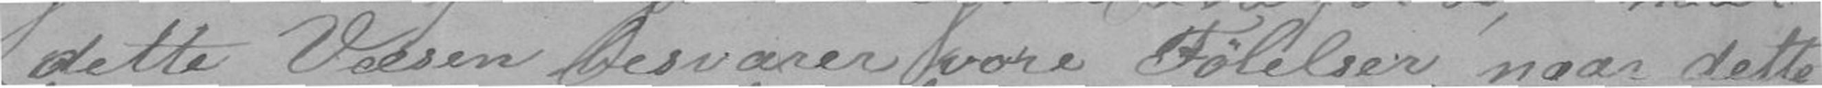dette Væsen besvarer vore Følelser, naar dette
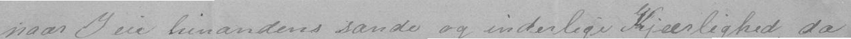naar I eie hinanden sande og inderlige Kjærlighed da
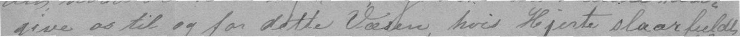give os til og for dette Væsen, hvis Hjerte slaar fuldt
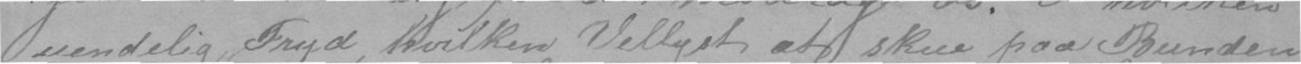uendelig Fryd, hvilken Vellyst at skue paa Bunden
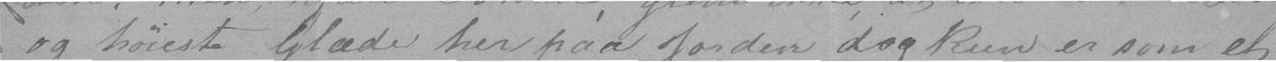og høieste Glæde her paa Jorden dog kun er som et
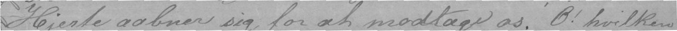Hjerte aabner sig for at modtage os. O! hvilken
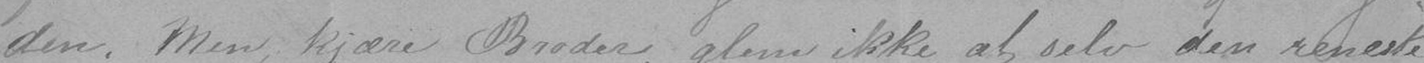den. Men kjære Broder, glem ikke, at selv den reneste
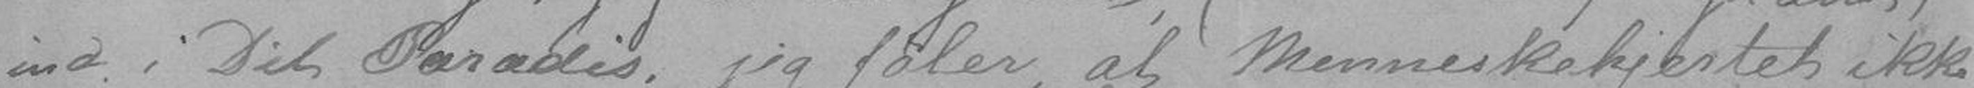ind i Dit Paradis; jeg føler, at Menneskehjertet ikke
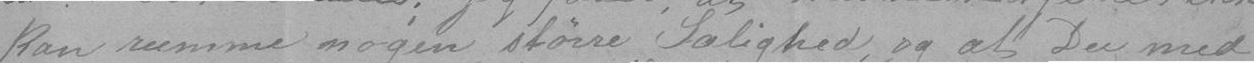kan rumme nogen større Salighed, og at Du med
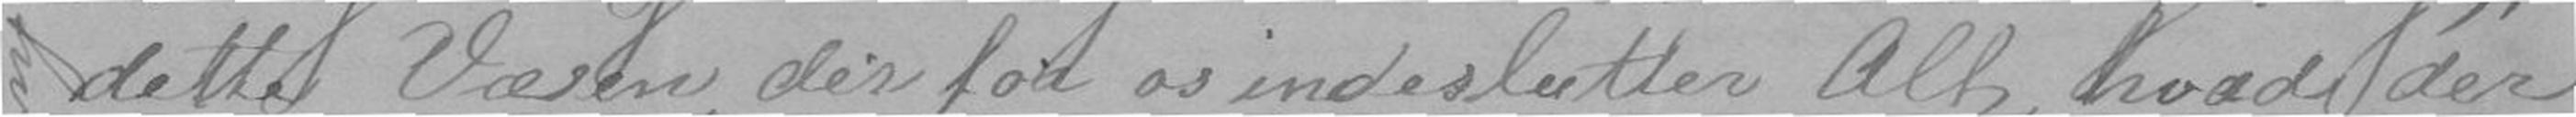dette Væsen der for os indeslutter Alt, hvad der
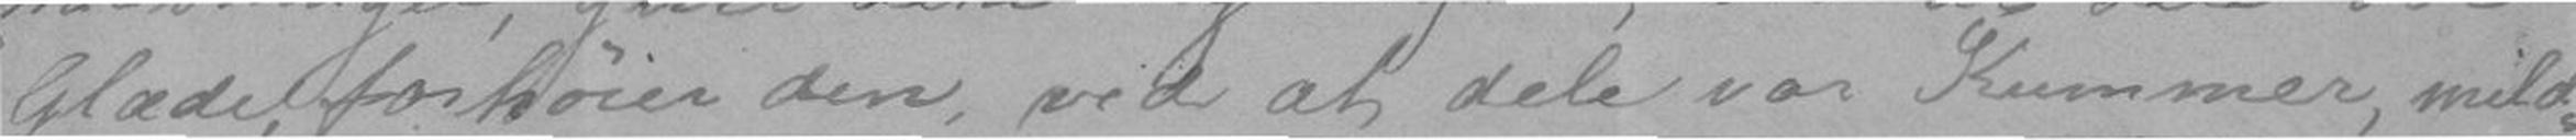Glæde, forhøier den, ved at dele vor Kummer, mild-
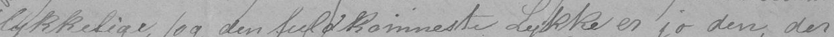lykkelige, (og den fuldkomneste Lykke er jo den, der
